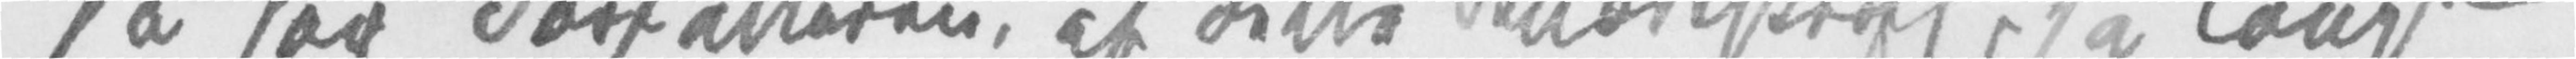så har Forfatteren af dette Smædeskrift, så langt
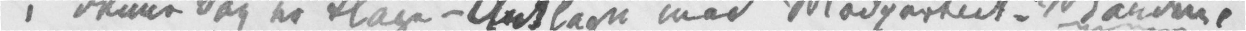i denne Sag er Klage-Anklage mod Modpartiet Manden,
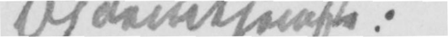Bjørnetjeneste.
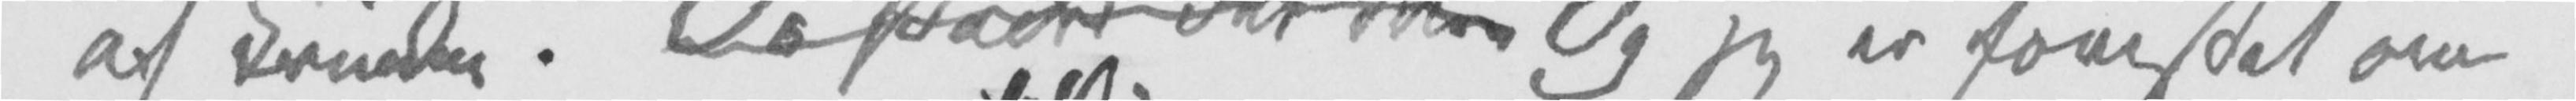af Kvinden. Os skade det ikke Og jeg er fo<gap />tet om
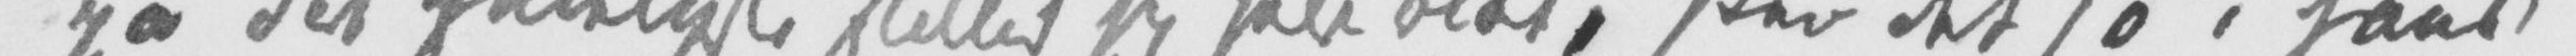på det g<gap />ligste stiller sig selv blot, sker det jo i hans
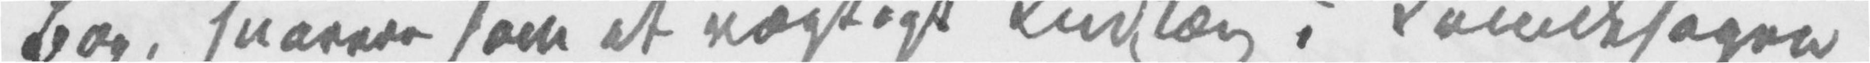Bog, snarere som et vægtigt Indlæg i Kvindesagen[.]
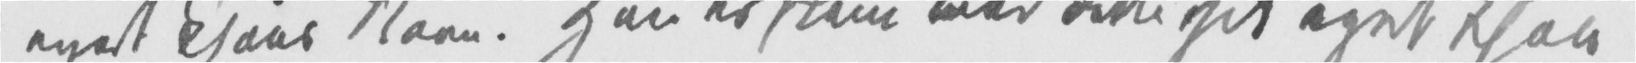eget Kjøns Navn. Han er slem mod dette sit eget Kjøn
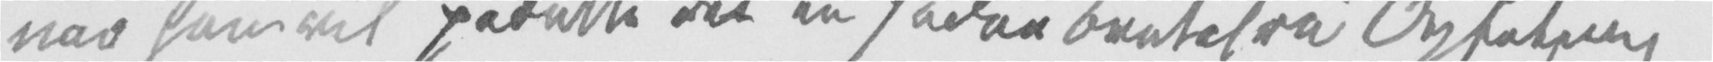når han vil pådutte det en sådan brutal rå Opfatning
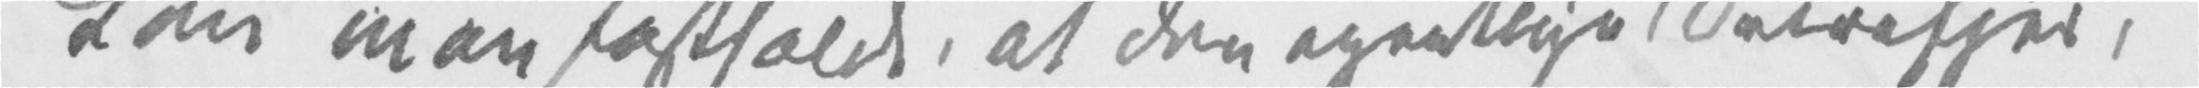Kan man fastholde, at den egentlige Drivefjer,
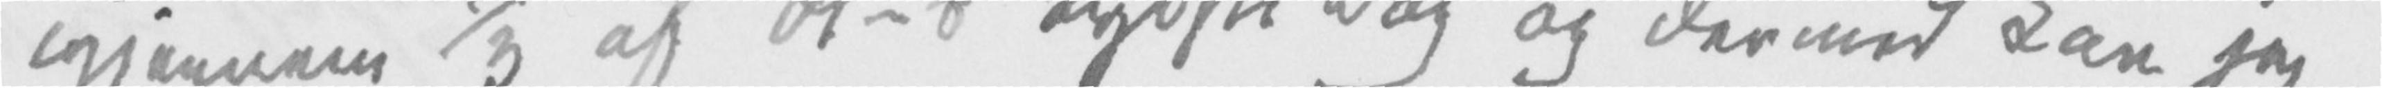igjennem ⅓ af St-s tydske Bog og dermed kan jeg
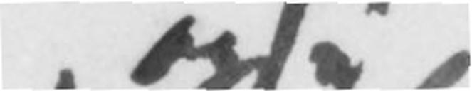også
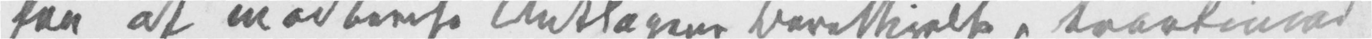hen at modbevise Anklagens Berettigelse, tvertimod
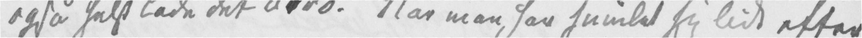også helst lade det bero. Når man har sundet sig lidt efter
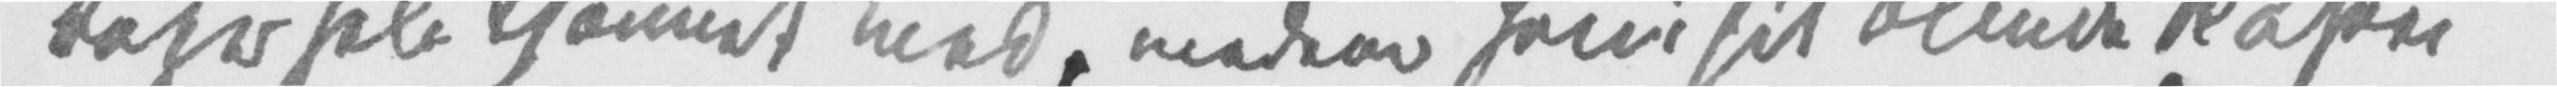tager hele Kjønnet med, medens han i sit blinde Raseri
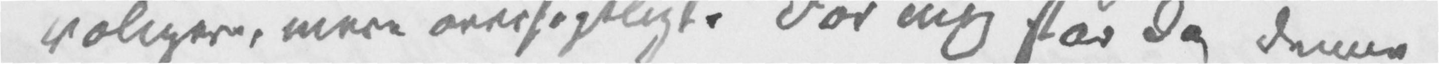roligere, mere oversigtligt. For mig står da denne
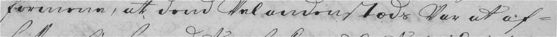formene, at dend Vel andenstæds Var at af-
